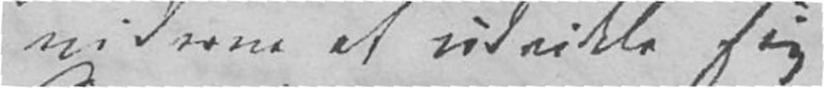viderne at udvikle sig
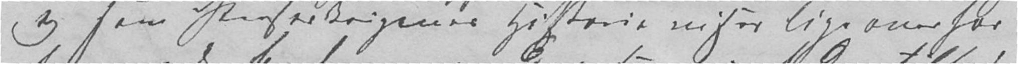og som Perserkrigenes Historie viser lige overfor
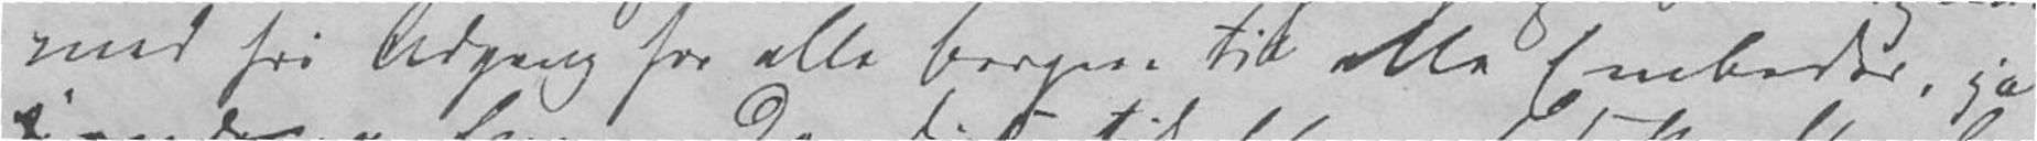med fri Adgang for alle Borgere til alle Embeder, ja
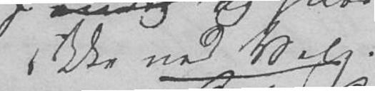ikke ved Valg.
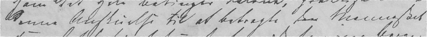denne Anskuelse til at betragte den Mennesket
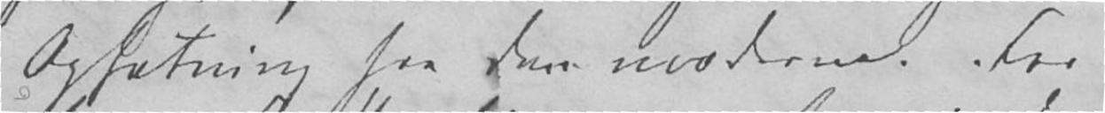Opfatning fra den moderne. For
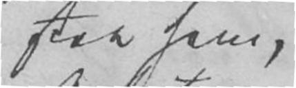staa ham,
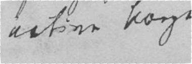active Borger
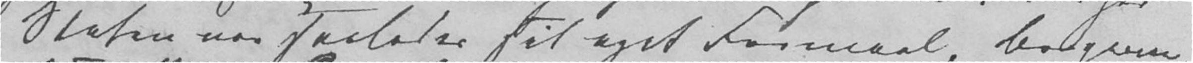Staten var saaledes sit eget Formaal, Borgerne,
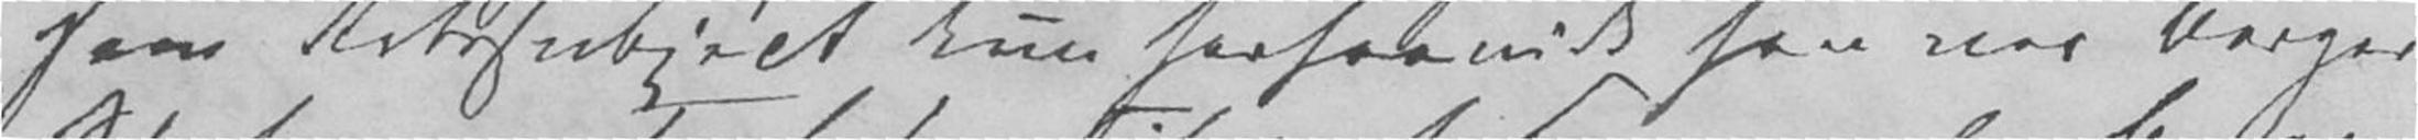som Retssubject kun forsaavidt han var Borger
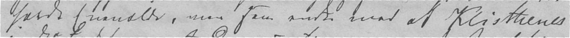havde Enevælde, men som endte med at Kleisthenes
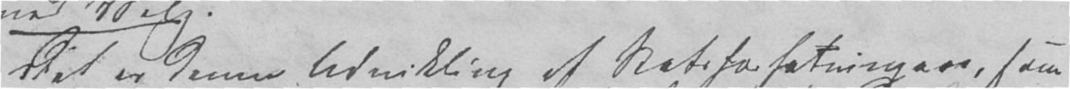Det er denne Udvikling af Statsforfatninger, som
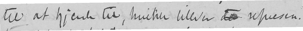til at kjende til, hvilke billder de represen-
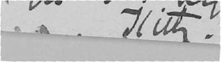Kitty.
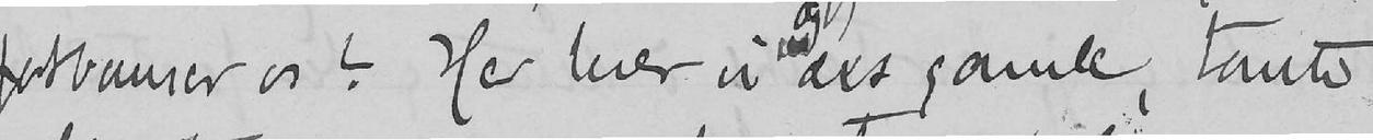forbauser os? Her lever vi ved det gamle, tante
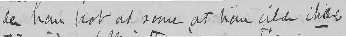de han blot at svare, at han vilde ikke
